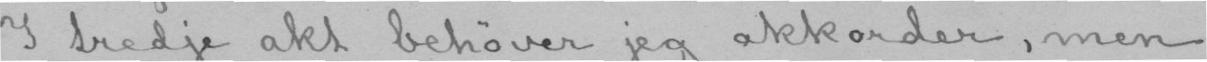I tredje akt behøver jeg akkorder, men
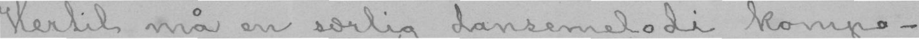Hertil må en særlig dansemelodi kompo-
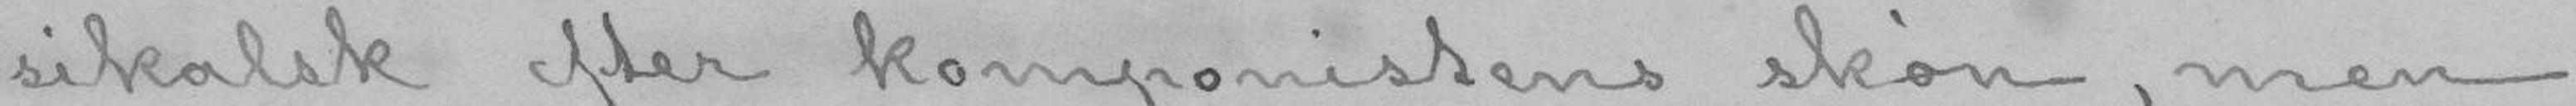sikalsk efter komponistens skøn, men
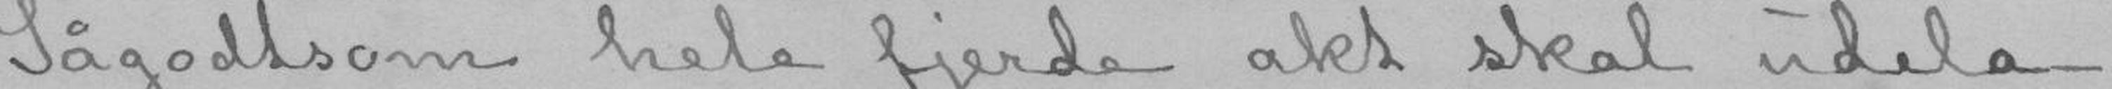Sågodtsom hele fjerde akt skal udela-
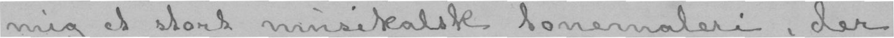mig et stort musikalsk tonemaleri, der
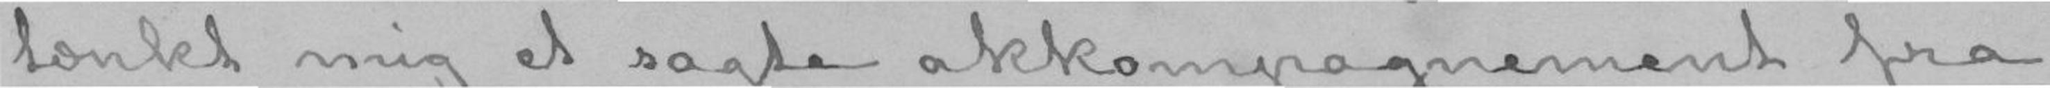tænkt mig et sagte akkompagnement fra
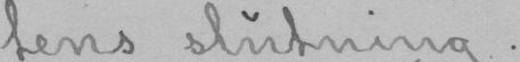tens slutning.
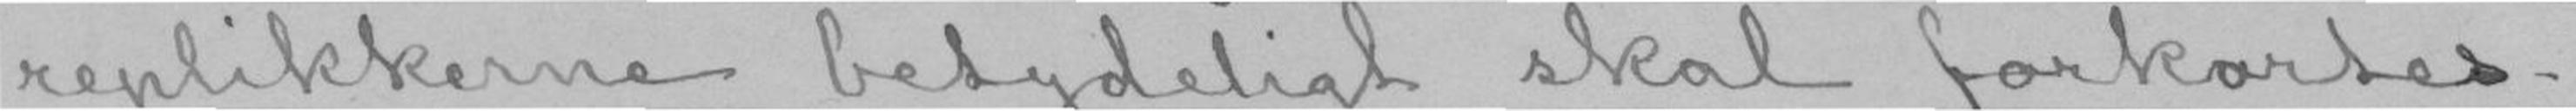replikkerne betydeligt skal forkortes.
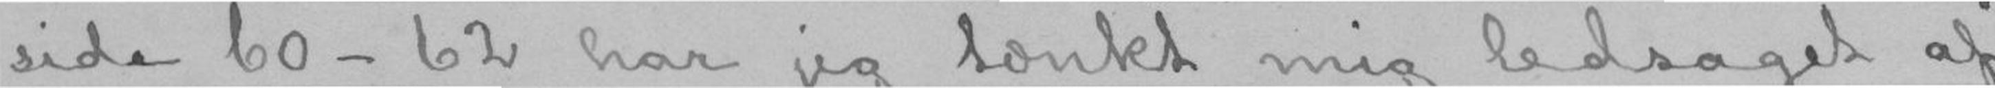side 60-62 har jeg tænkt mig ledsaget af
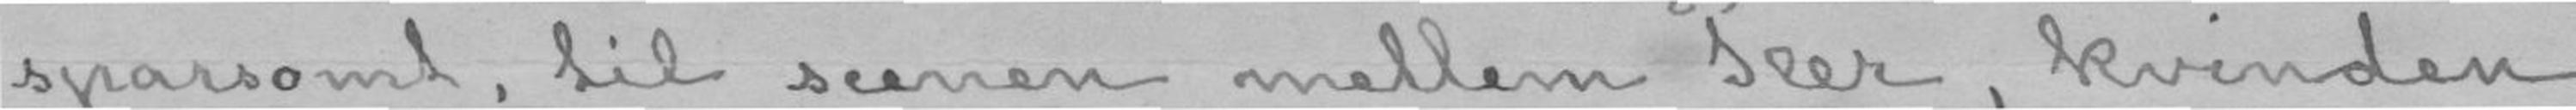sparsomt, til scenen mellem Peer, kvinden
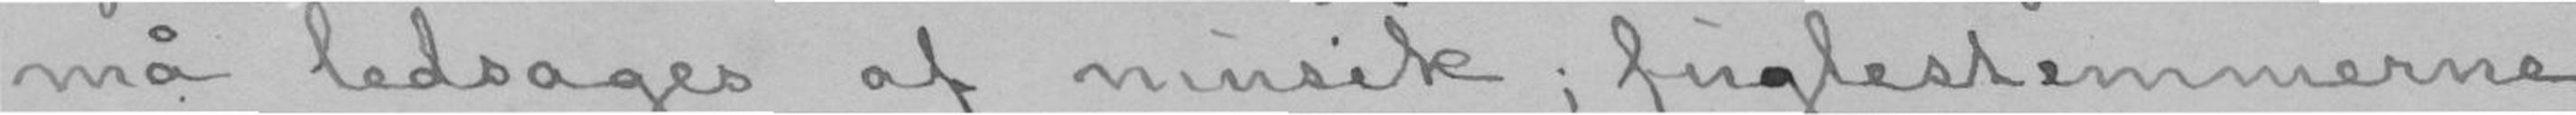må ledsages af musik; fuglestemmerne
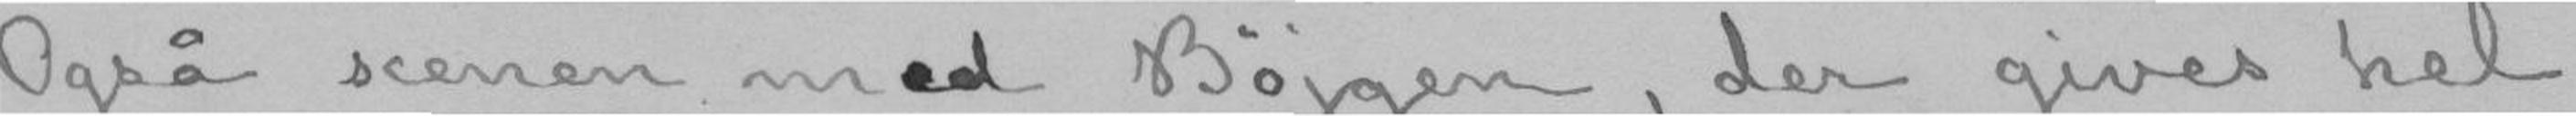Også scenen med Bøjgen, der gives hel,
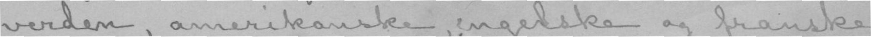verden, amerikanske, engelske og franske
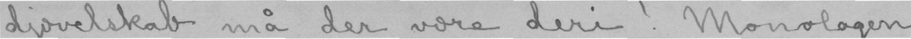djævelskab må der være deri! Monologen
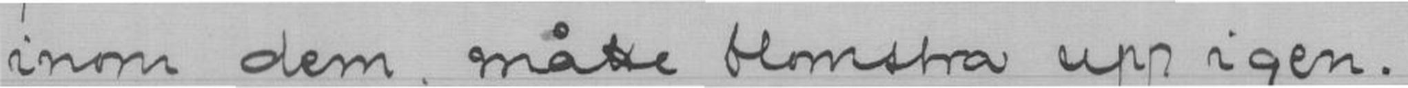inom dem måtte blomstra upp igen.
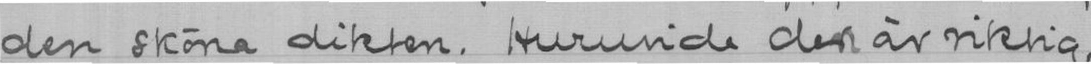den sköna dikten. Huruvide den är riktig,
